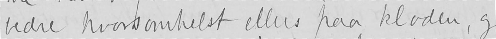bedre, hvorsomhelst ellers paa kloden, og
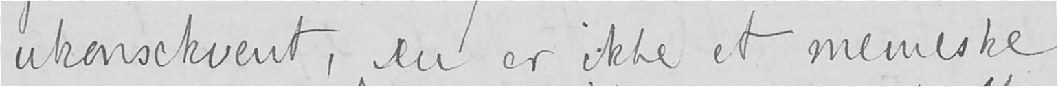ukonsekvent, "Du er ikke et menneske
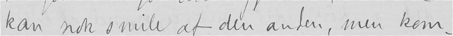kan nok smile af den anden, men kom-
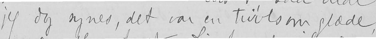jeg dog synes, det var en tvivlsom glæde,
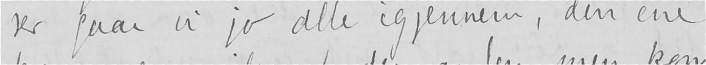ser faar vi jo alle igjennem, den ene
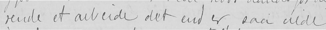rende et arbeide det end er, saa vilde
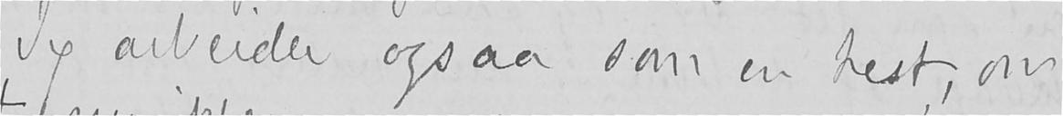Jeg arbeider ogsaa som en hest, om
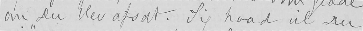om Du blev afsat. Sig hvad vil Du
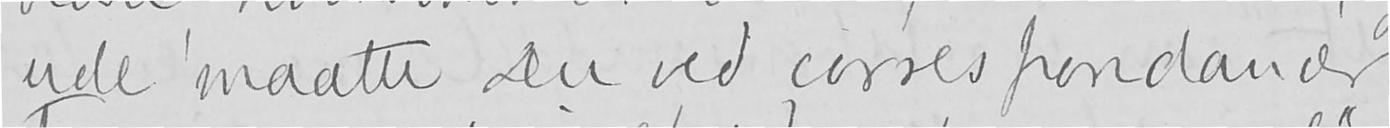ude maatte Du ved correspondancer
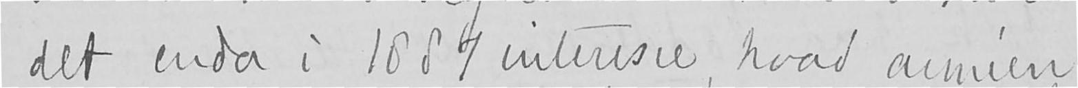det enda i 1887 interesse, hvad arméen
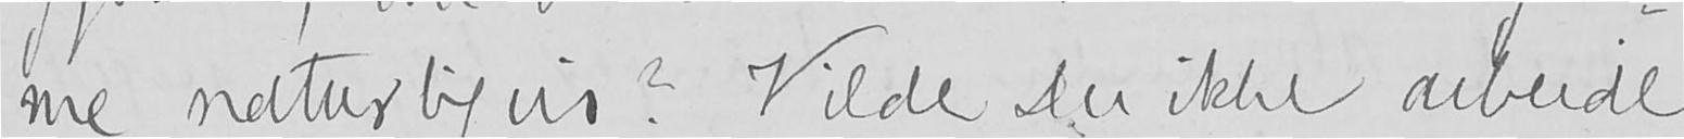me naturligvis? Vilde Du ikke arbeide
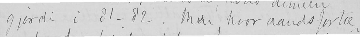gjorde i 81-82. Men hvor aandsfortæ-
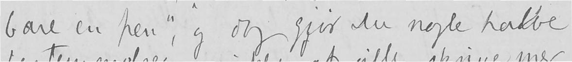bare en pen", og dog gjør Du nogle halve
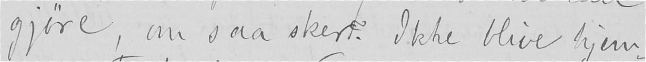gjøre, om saa sker? Ikke blive hjem-
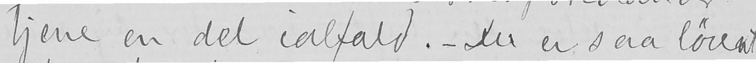tjene en del ialfald. - Du er saa løient
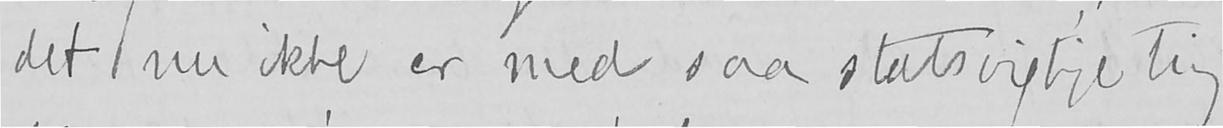det nu ikke er med saa statsvigtige ting
